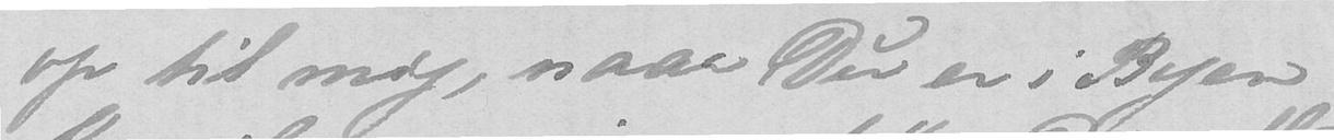op til mig, naar Du er i Byen
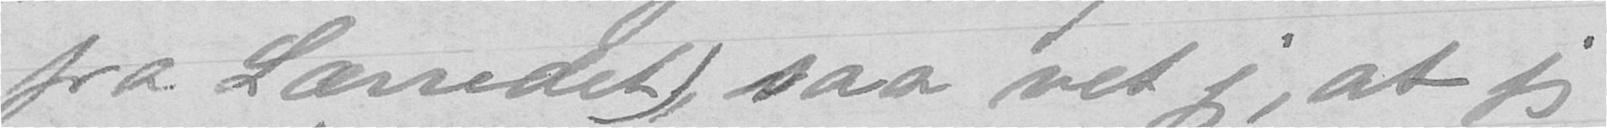fra Lærredet), saa vet jeg, at jeg
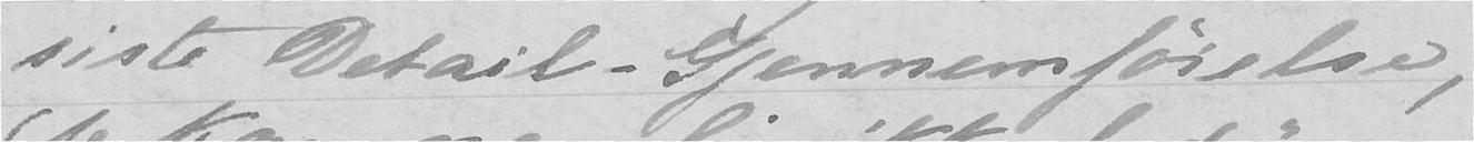siste Detail-Gjennemførelse,
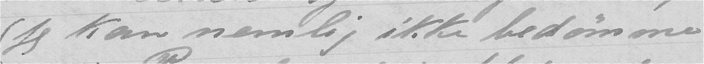(jeg kan nemlig ikke bedømme
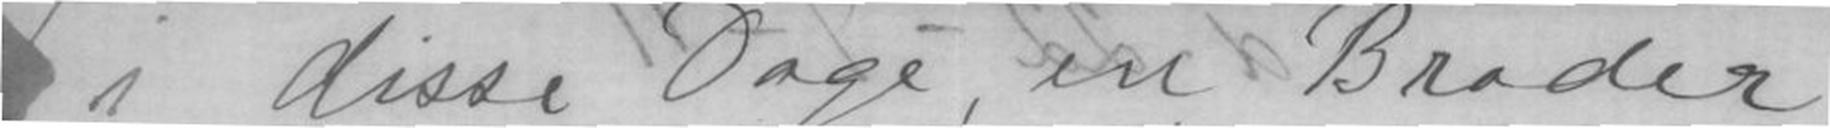i disse Dage, en Broder
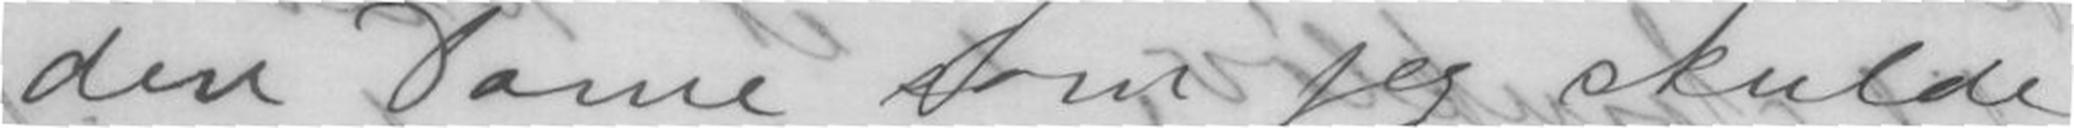den dame som jeg skulde
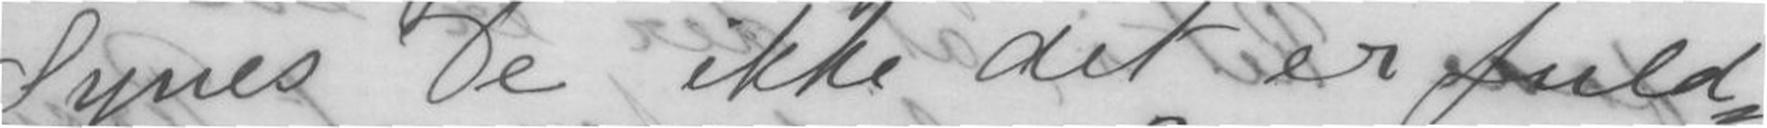Synes De ikke det er fuld-
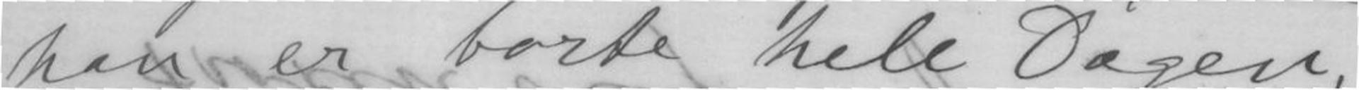han er borte hele Dagen,
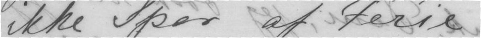ikke Spor af Ferie.
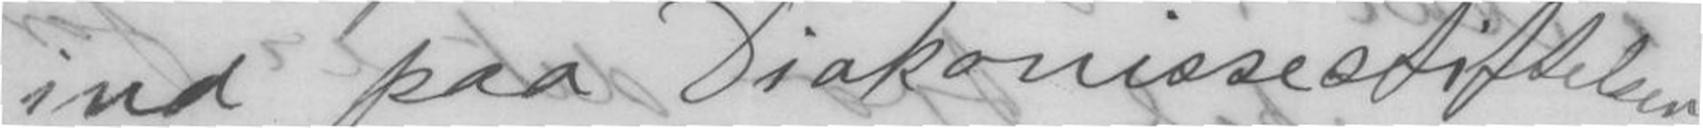ind paa Diakonissestiftelsen
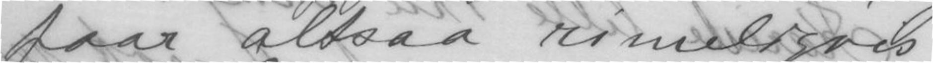faar altsaa rimeligvis
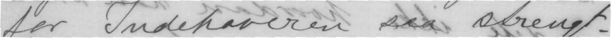for Indehaveren saa strengt.
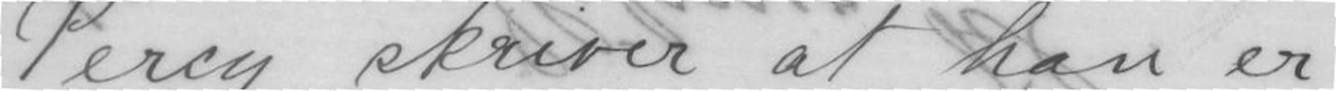Percy skriver at han er
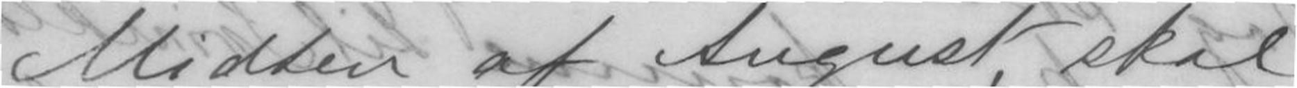Midten af August, skal
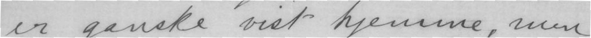er ganske vist hjemme, men
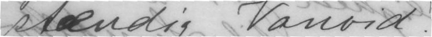stændig Vanvid?
